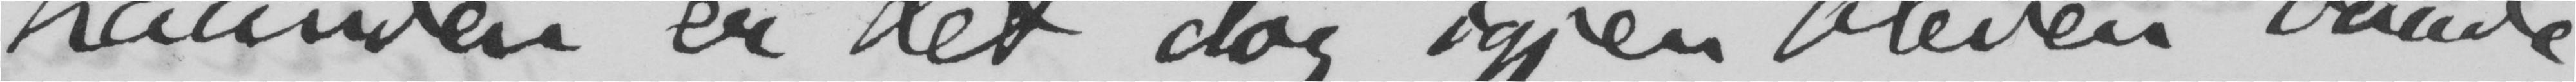haanden er det dog igjen bleven baade
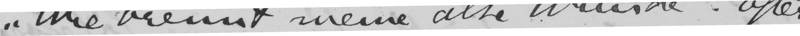«Wie brennt meine alte Wünde»! Efter-
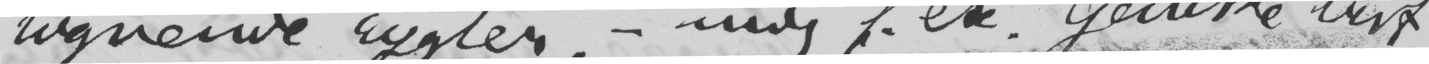lignende rygter, mig f.ex. Ganske vist,
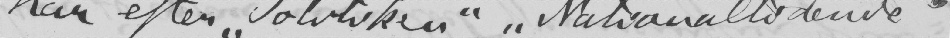har efter «Politiken», «Nationaltidende»
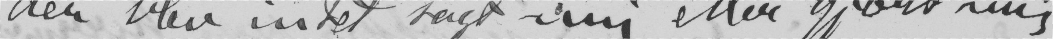der blev intet sagt mig eller gjort mig,
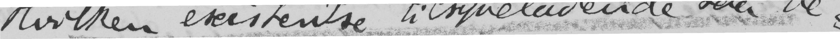Hvilken existentse tilsyneladende saa be-
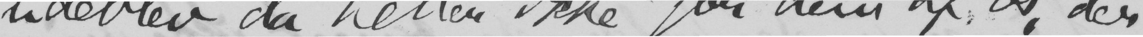udeblev da heller ikke for dem af os, der
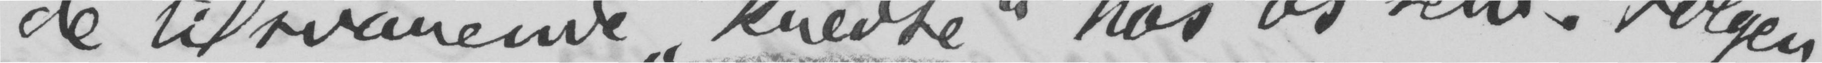de tilsvarende «kredse» hos os selv. Følgen
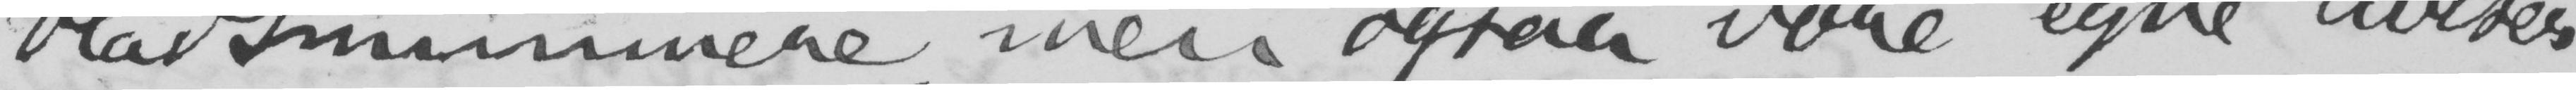bladsnummere, men ogsaa vore egne aviser
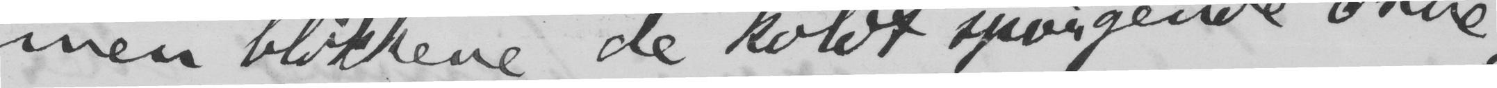men blikkene, de koldt spørgende øine,
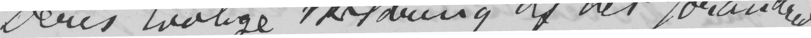Deres livlige skildring af det forandrede
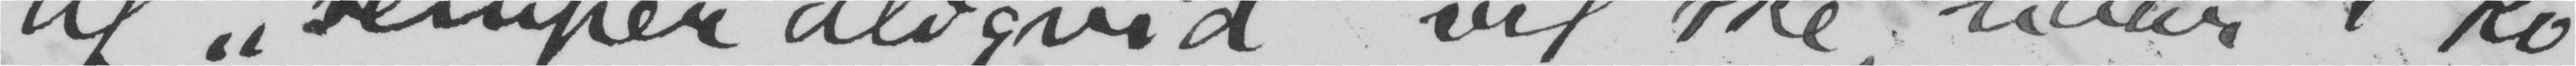af «semper aliqvid» vil ske, naar i Kø-
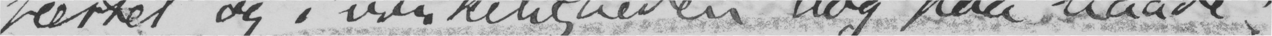fæstet og i virkeligheden dog paa naade!
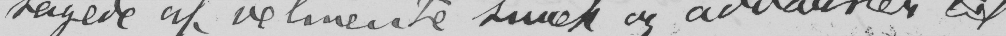sagede af velmente smek og advarsler til
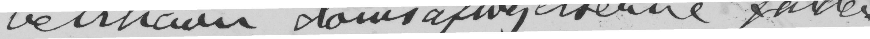benhavn domsafsigelserne falder.
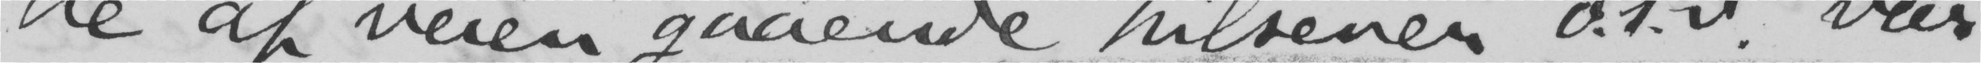de af veien gaaende hilsener o.s.v var
